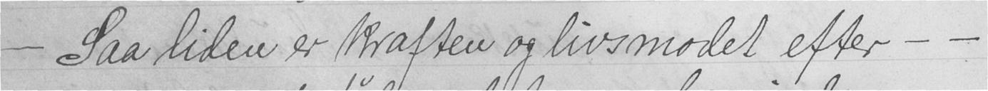- Saa liden er kraften og livsmodet efter - -
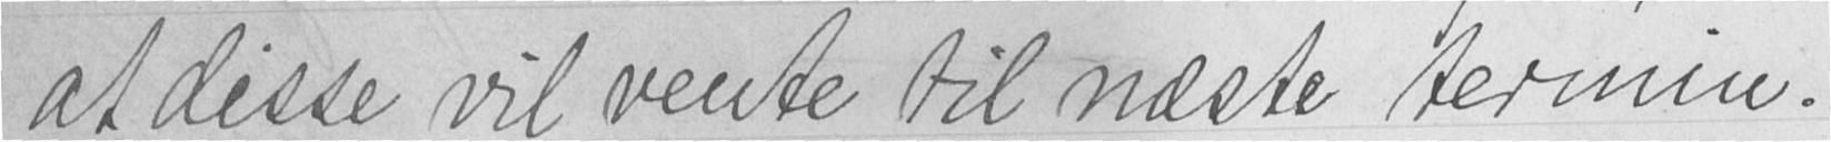at disse vil vente til næste termin.
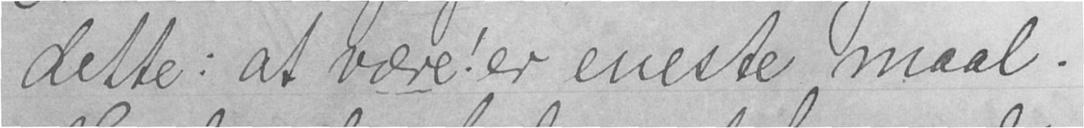dette: at være! er eneste maal.
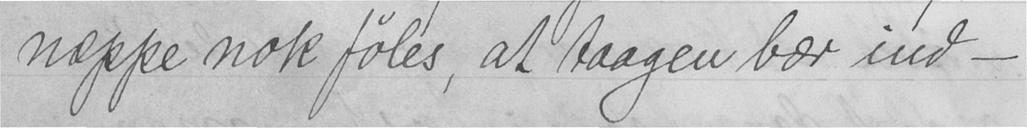næppe nok føles, at taagen bær ind -
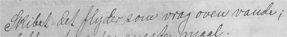Skibet det flyder som vrag oven vande;
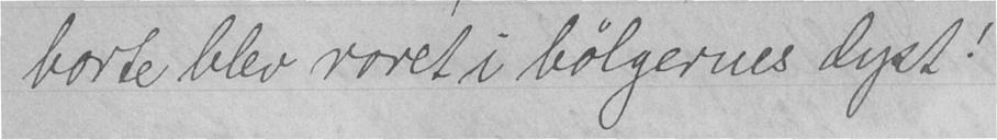borte blev roret i bølgernes dyst!
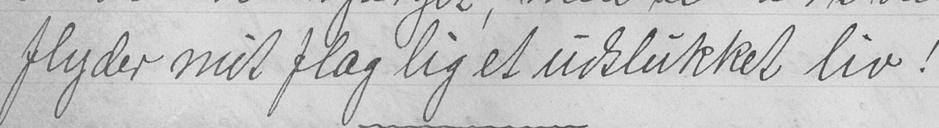flyder mit flag lig et udslukket liv!
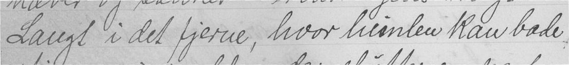Langt i det fjerne, hvor himlen kan bade
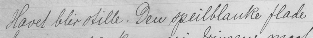Havet blir stille. Den speilblanke flade
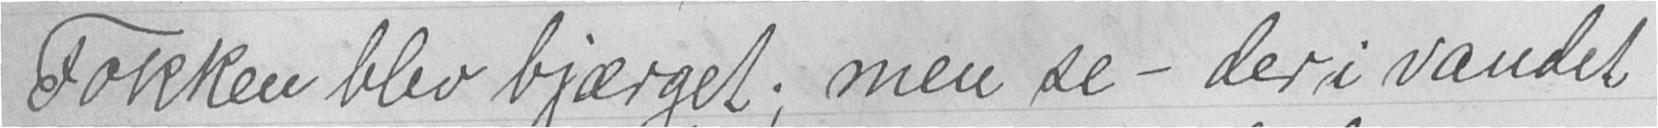Fokken blev bjærget; men se - der i vandet
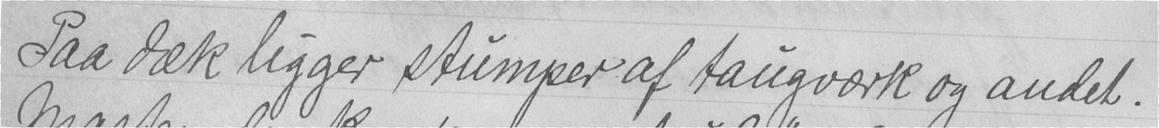Saa dæk ligger stumper af taugværk og andet.
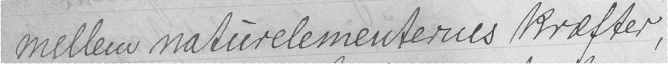mellem naturelementernes kræfter,
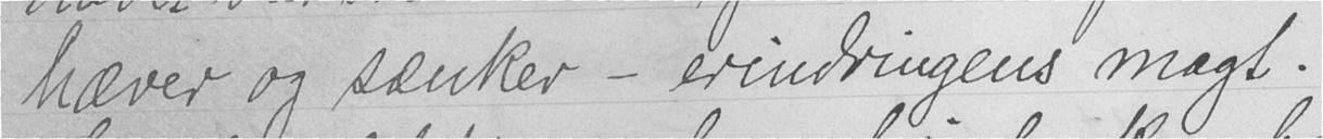hæver og sænker - erindringens magt.
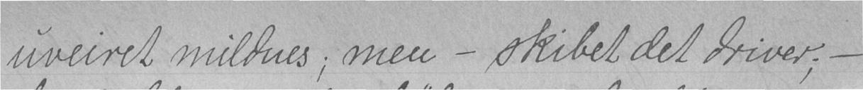uveiret mildnes; men - skibet det driver; -
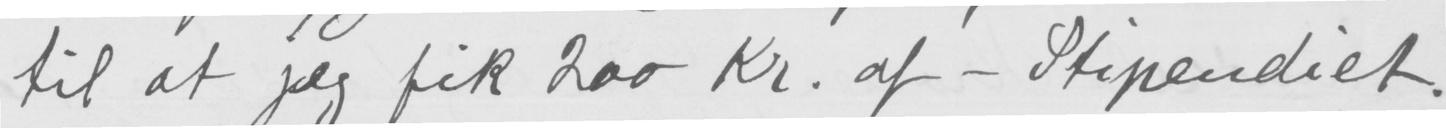til at jæg fik 200 Kr. af — Stipendiet.
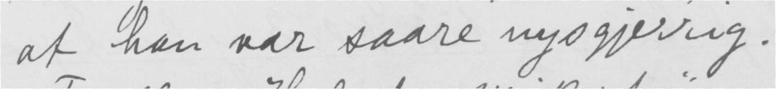at han var saare nysgjerrig.
